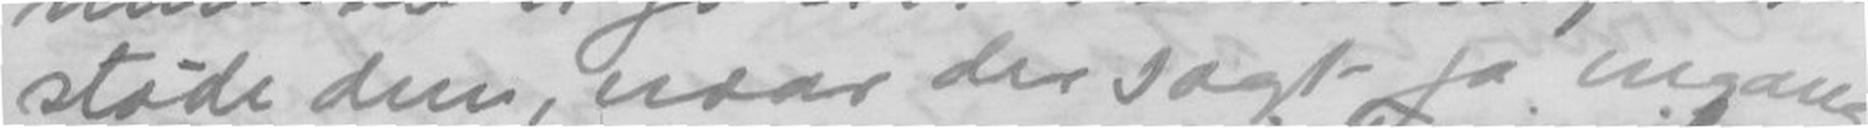støde dem, naar der sagt ja engang.
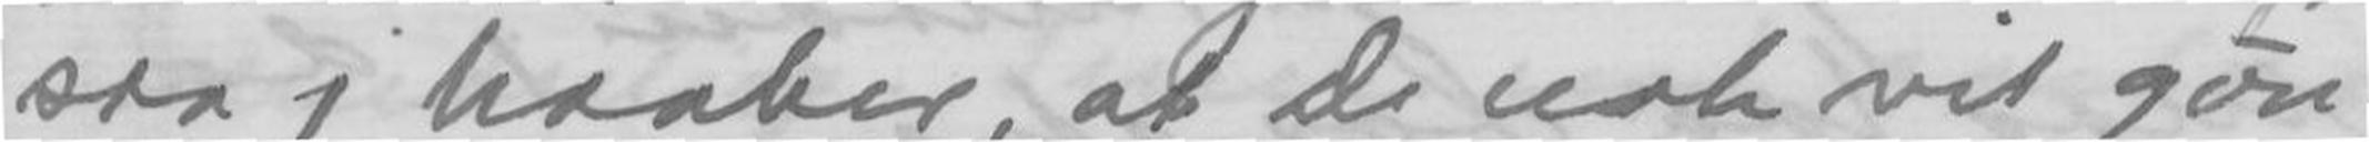saa j haaber, at de nok vil gøre
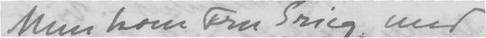Men kaere Fru Grieg med
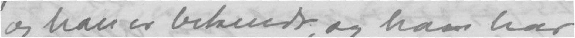og han er bekendt, og han har
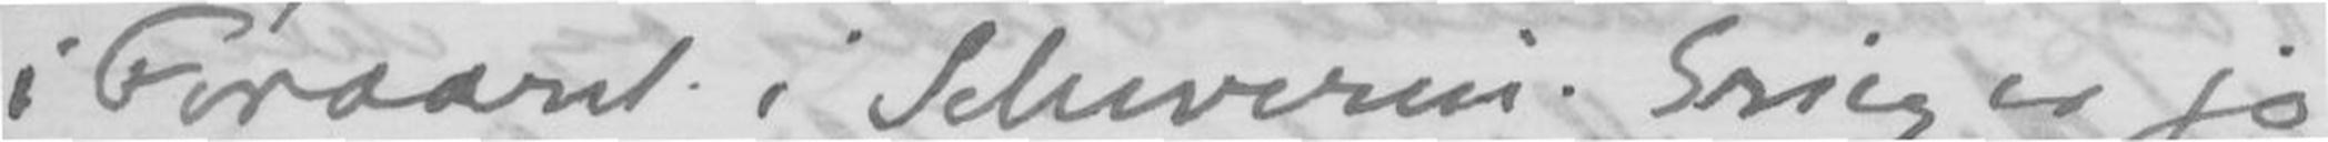i Foraaret i Schwerin. Grieg er jo
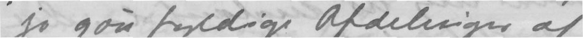j jo gøre fyldige Afdelinger af
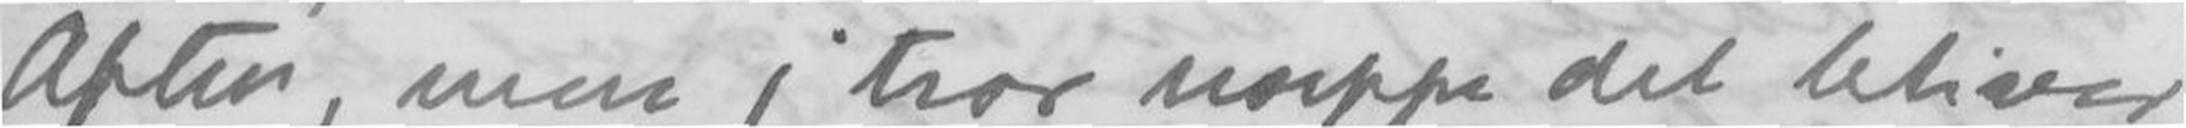Aften, men j tror naeppe det bliver
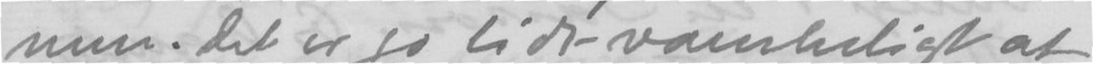men. Det er jo lidt vanskeligt at
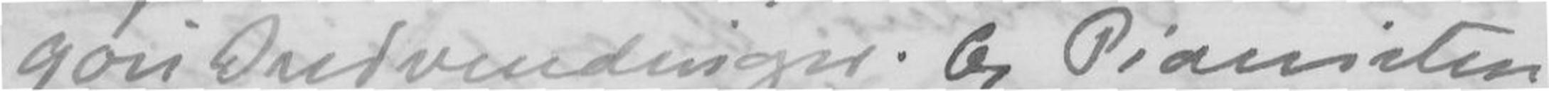gøre Indvendinger. Og Pianisten
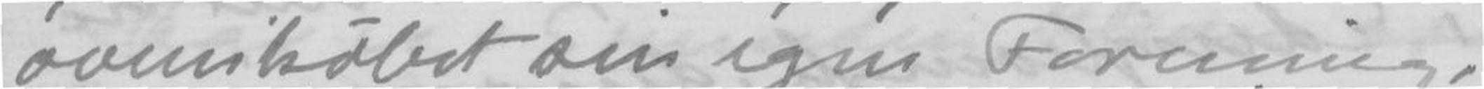ovenikøbet sin egen Forening,
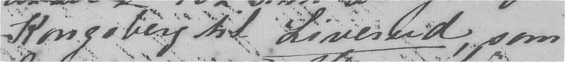Kongsberg til Liverud, som
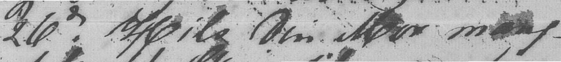26de. Hils Din Mor mang-
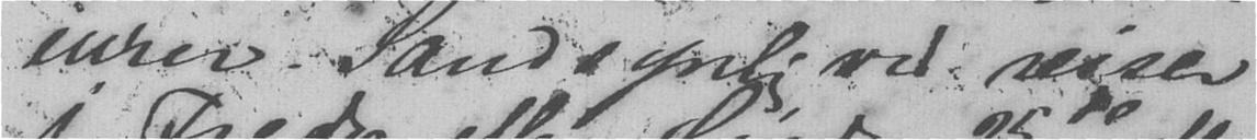eurer. Sandsynligvis reiser
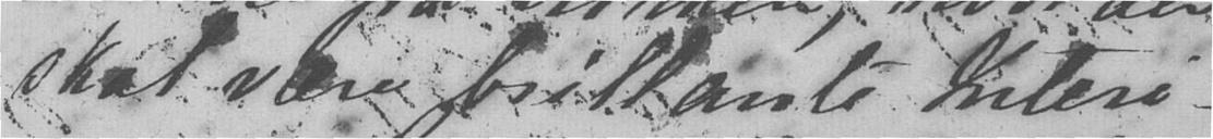skal være brillante Interi-
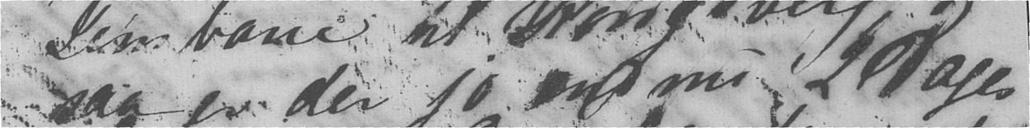saa er der jo endnu 2 Dages
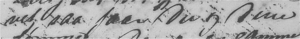vet, saa faar Du og Dine
